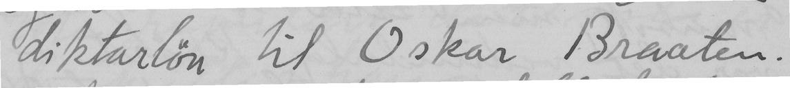diktarlön til Oskar Braaten.
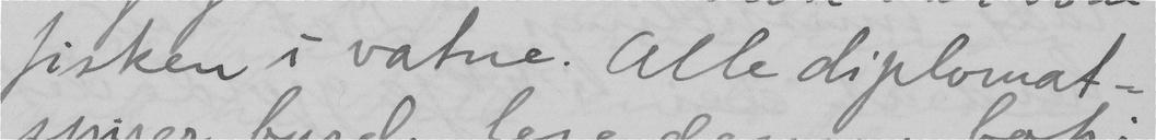fisken i vatne. Alle diplomat-
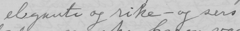elegante og rike – og sers
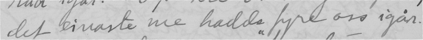det einaste me hadde fyre oss igår.
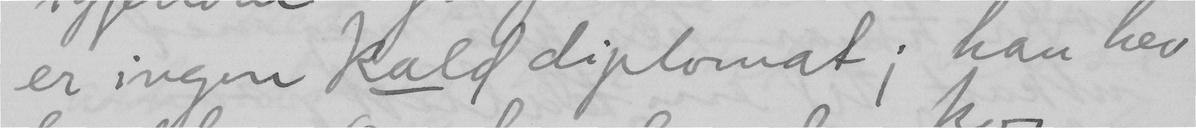er ingen kald diplomat; han hev
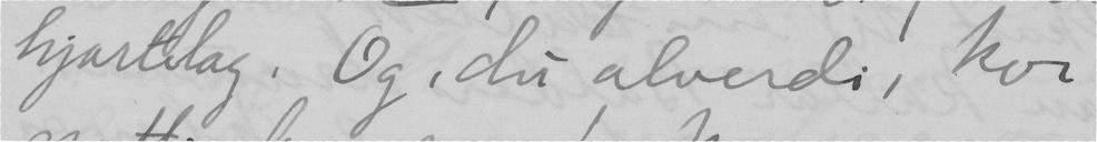hjartelag. Og, du alverdi, kor
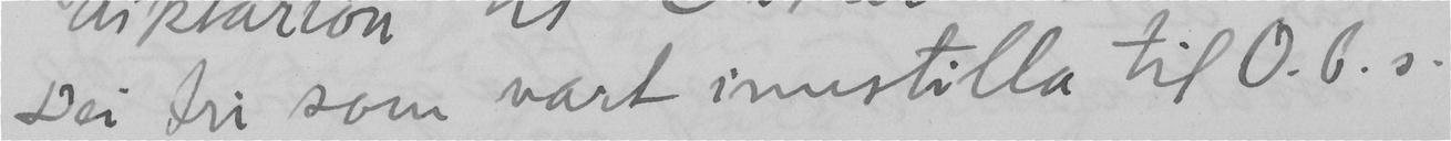Dei tri som vart innstilla til O.G.s.
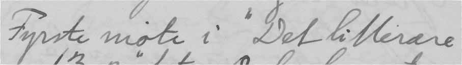Fyrste möte i Det litterære
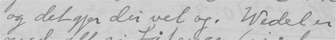og det gjer dei vel og. Wedel er
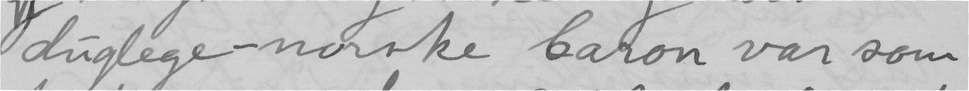duglege – norske baron var som
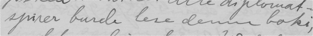spirer burde lese denne boki,
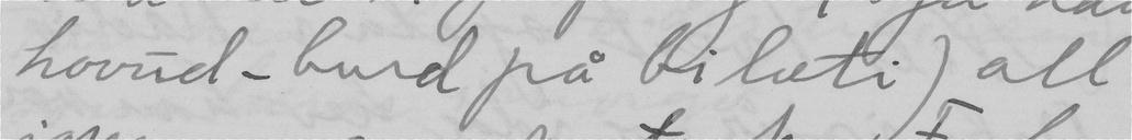hovud-burd på bilæti) all
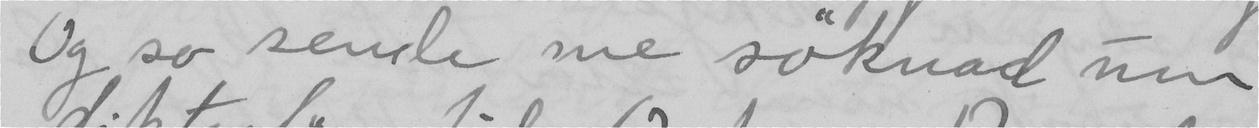Og so sende me söknad um
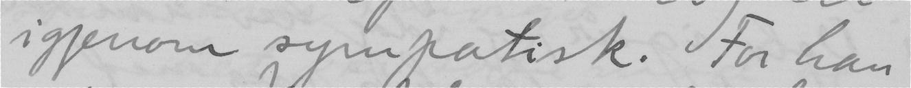igjenom sympatisk. For han
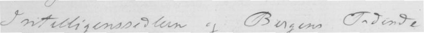Intelligenssedlern og Bergens Tidende
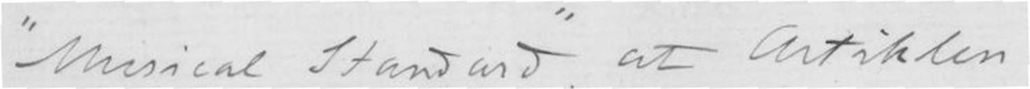i "Musical Standard", at Artiklen
